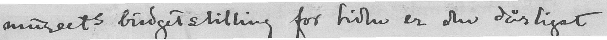museets budgetstilling for tiden er den dårligst
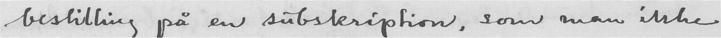bestilling på en subskription, som man ikke
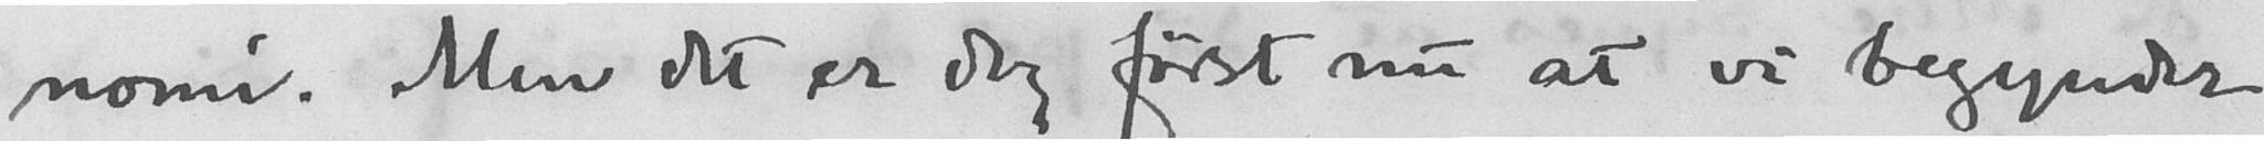nomi. Men det er dog først nu at vi begynder
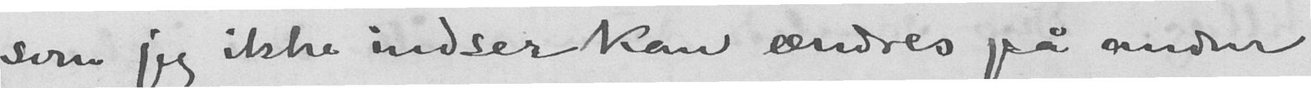som jeg ikke indser kan ændres på anden
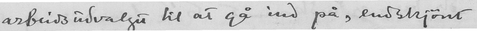arbeidsudvalget til at gå ind på, endskjønt
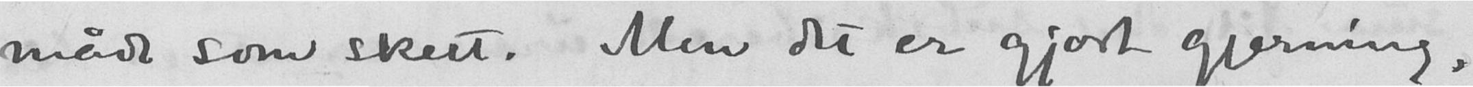måde som skeet. Men det er gjort gjerning,
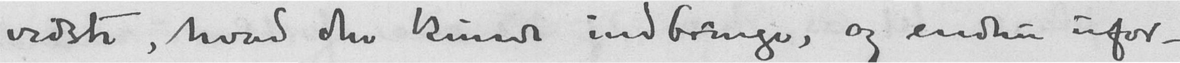vidste, hvad der kunde indbringe, og endnu ufor-
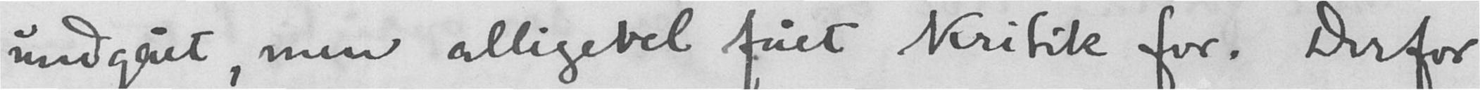undgået, men alligevel fået kritik for. Derfor
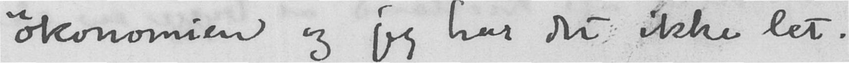økomomien og jeg har det ikke let.
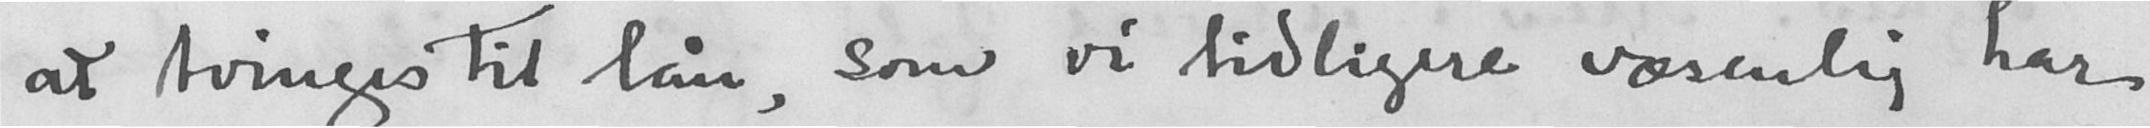at tvinges til lån, som vi tidligere væsenlig har
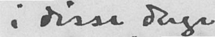i disse dage
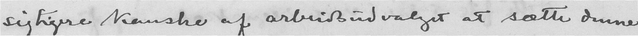sigtigere kanske af arbeidsudvalet at sætte denne
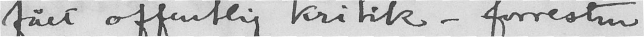fået offentlig kritik - forresten
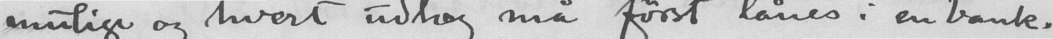mulige og hvert udlæg må først lånes i en bank.
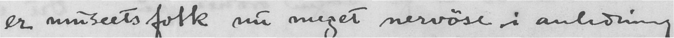er museets folk nu meget nervøse i anledning
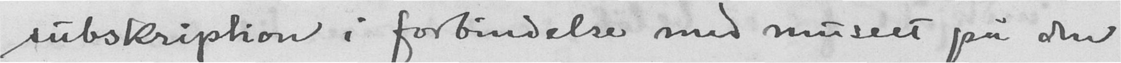subskription i forbindelse med museet på den
